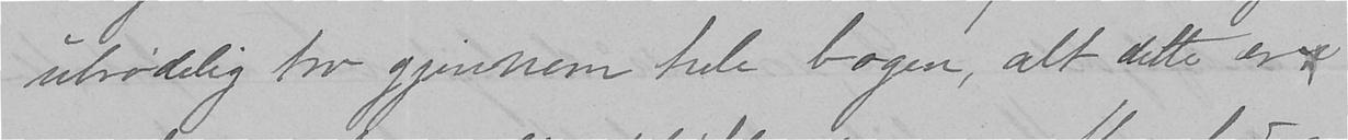ubrødelig tro gjennom hele bogen, alt dette er i
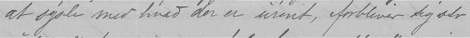at sysle med hvad der er urent, forbliver sig selv
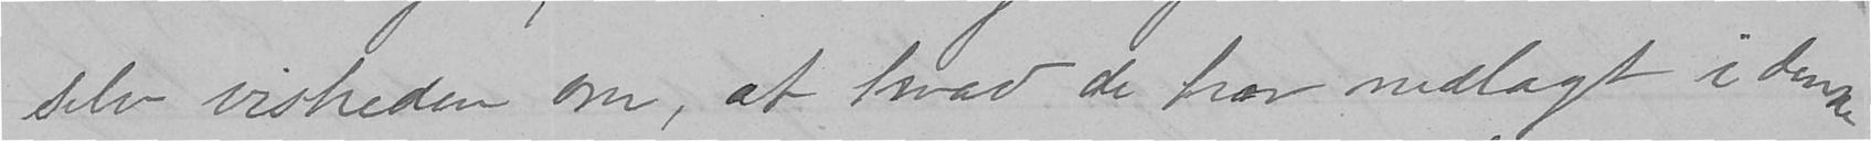selv visheden om, at hvad de har nedlagt i denne
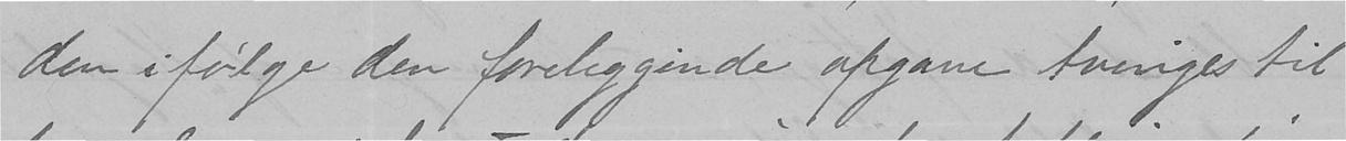den ifølge den foreliggende opgave tvinges til
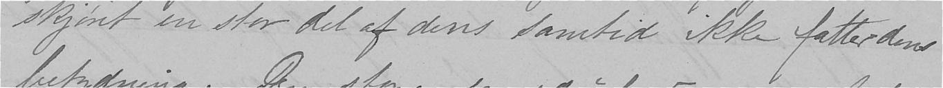skjønt en stor del af dens samtid ikke fatter dens
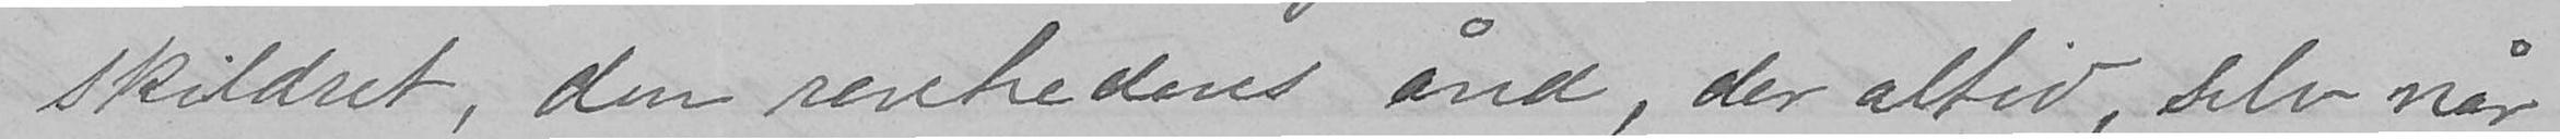skildret, den renhedens ånd, der altid, selv når
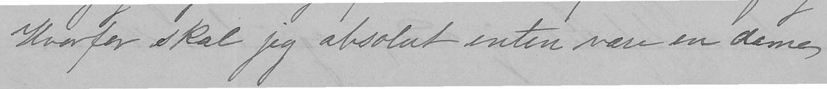Hvorfor skal jeg absolut enten være en dame,
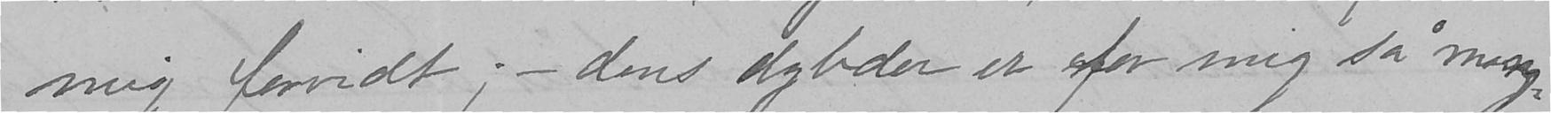mig forvidt; - dens dybder er for mig så mang-
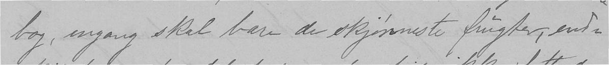bog, engang skal bære de skjønneste frugter, end-
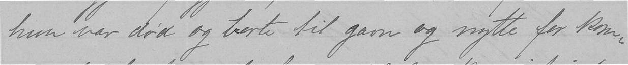hun var død og borte til gavn og nytte for kom-
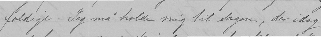foldige. Jeg må holde mig til sagen, der idag
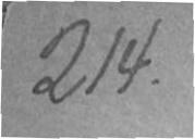214.
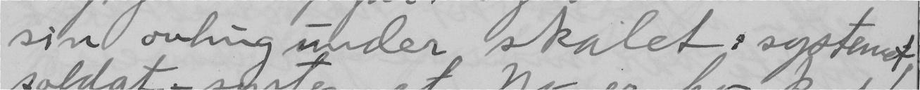sin ovlung under skalet: systemet,
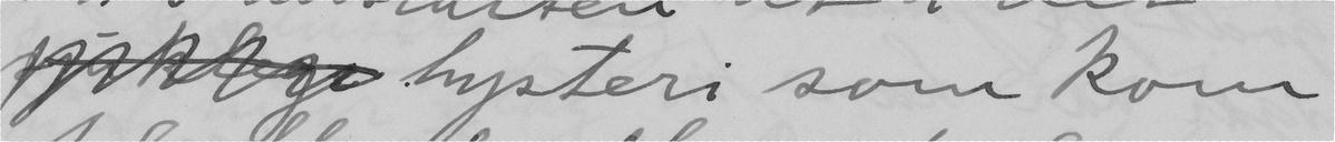hysteri som kom
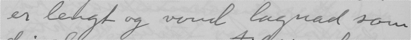er lengt og vond lagnad som
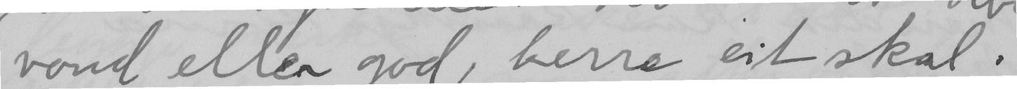vond eller god, berre eit skal.
